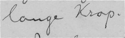lange Krop.
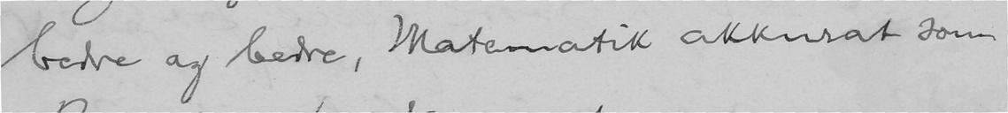bedre og bedre, Matematik akkurat som
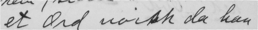et Ord norsk da han
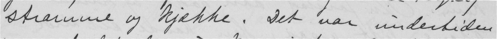stramme og kjække. Det var undertiden
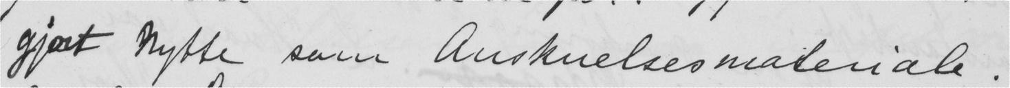gjot nytte som Anskuelsesmateriale.
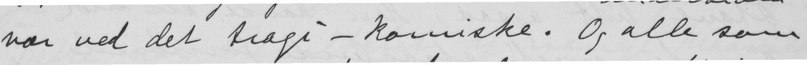var ved det tragi-komiske. Og alle som
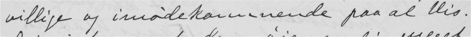villige og imødekommende paa al Vis.
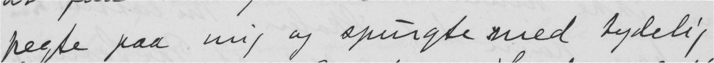pegte paa mig og spurgte med tydelig
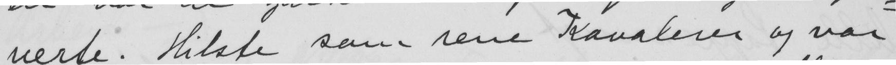nerte. Hilste som rene Kavalerer og var
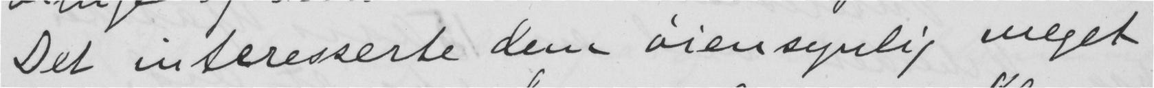Det interesserte dem øiensynlig meget
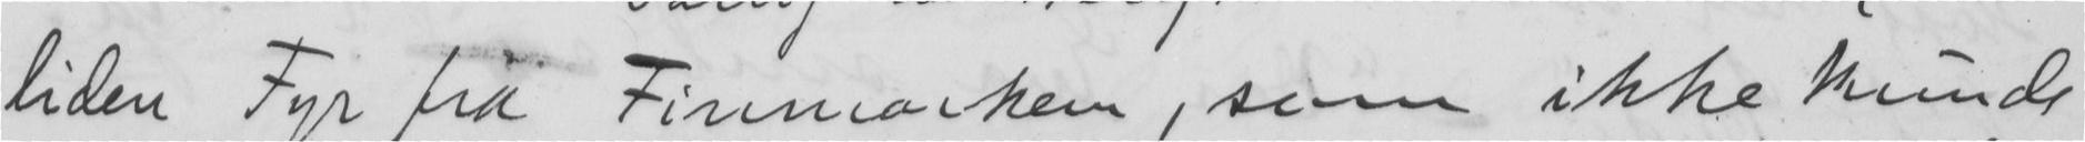liden Fyr fra Finmarken, som ikke Kunde
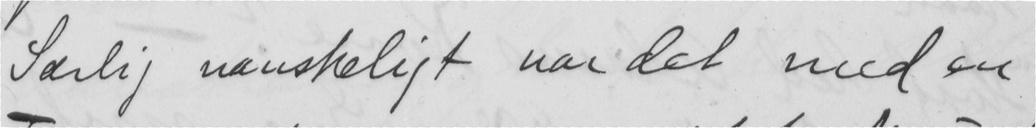Særlig vanskeligt var det med en
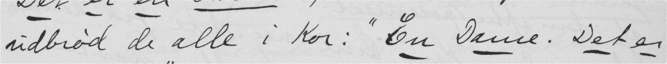udbrød de alle i Kor. "En Dame. Det er
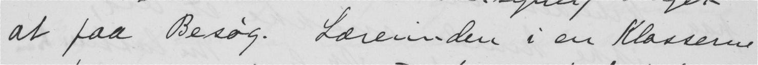at faa Besøg. Lærerinden i en Klasserne
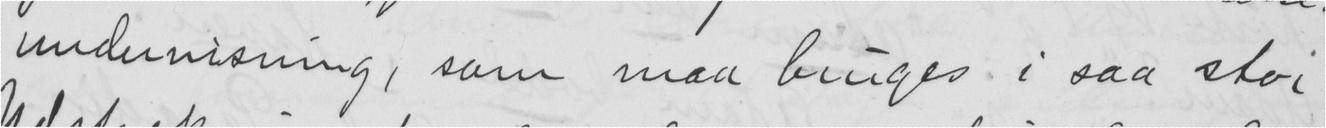undervisning, som maa bruges i saa stor
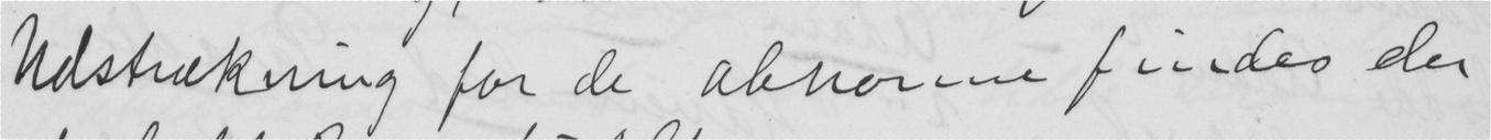Udstrækning for de abnormee findes der
